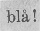blå!
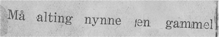Må alting nynne en gammel
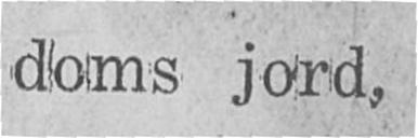doms jord,
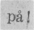på!
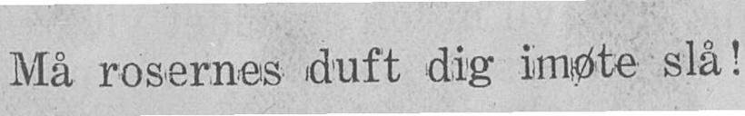Må rosernes duft dig imøte slå!
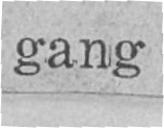gang - - -
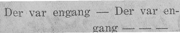Der var engang - Der var en-
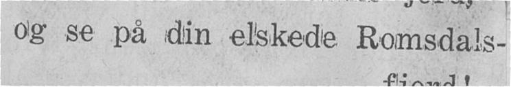og se på din elskede Romsdals-
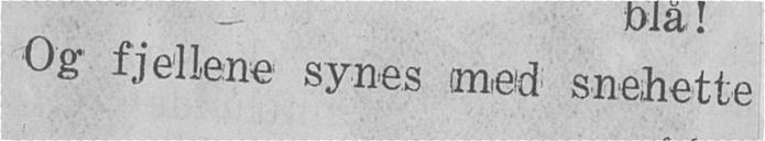Og fjellene synes med snehette
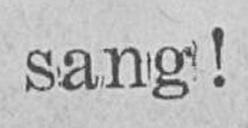sang!
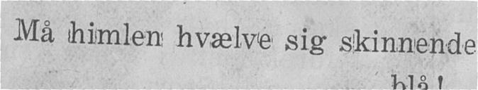Må himlen hvælve sig skinnende
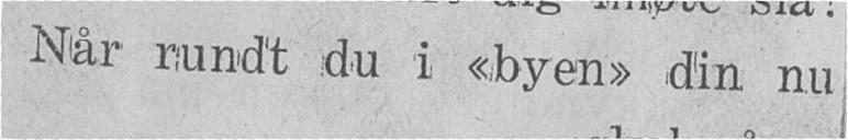Når rundt du i «byen» din nu
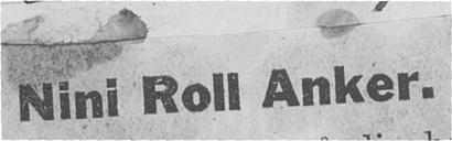Nini Roll Anker.
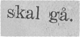skal gå.
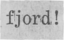fjord!
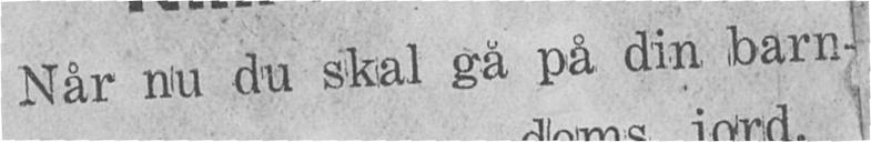Når nu du skal gå på din barn-
